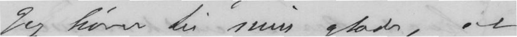Jeg hører til min glæde, at
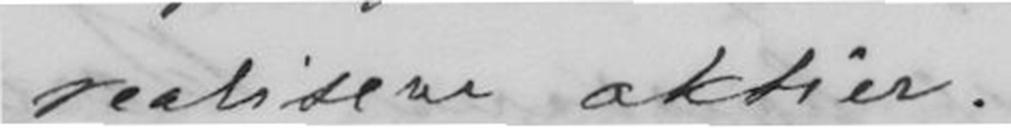realisaere aktier.
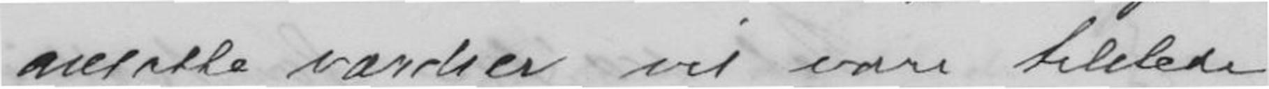antatte værdier vil være tilstede
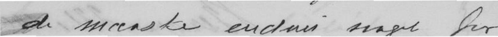de maske endnu for
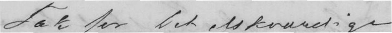Tak for Det elskværdige
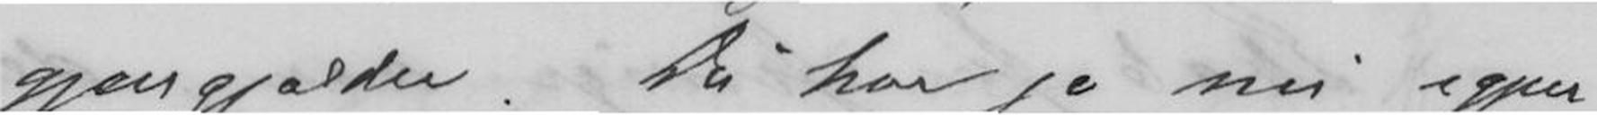gjengjælder. Du har jo nu igjen
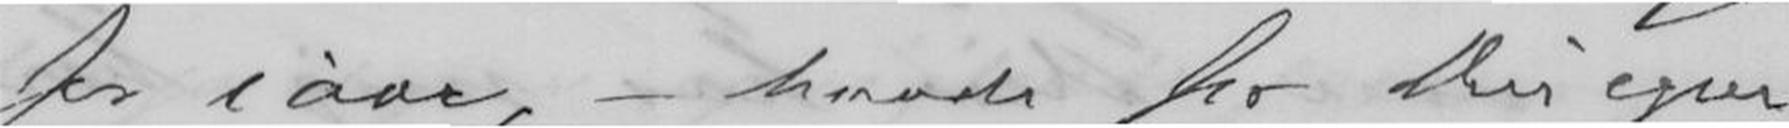for iaar, - havde for Din egen
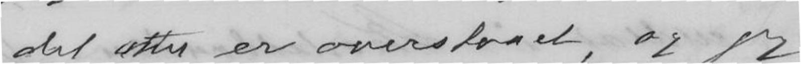det atter er overstaaet, og jeg
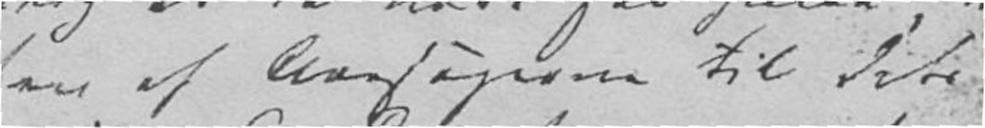en af Aarsagerne til dets
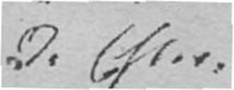Alle de Efter-
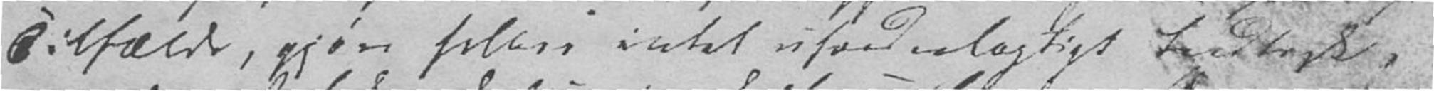Tilfælde, gjøre hellere intet ufordelagtigt Indtryk,
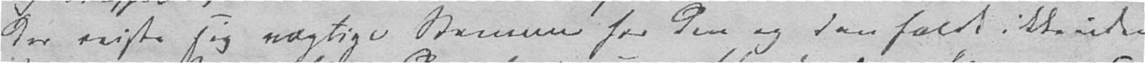der viste sig vægtige Stemmer for den og den faldt ikke uden
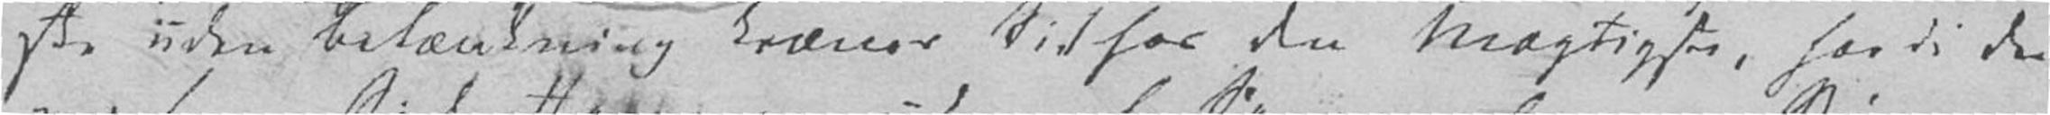ste uden Betænkning kræver Sit hos den Mægtigste, fordi der
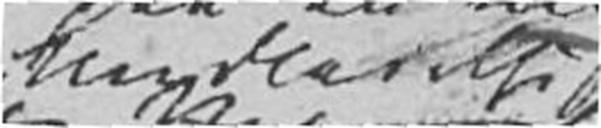Undladelse
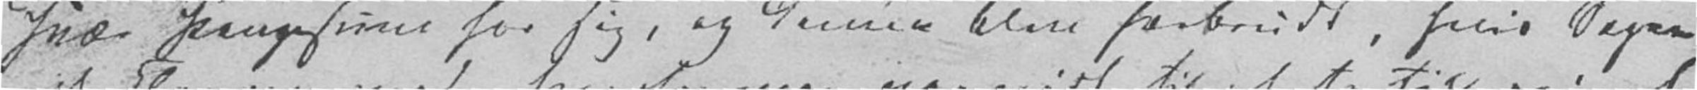stor Pengesum for sig, og denne blev forbrudt, hvis Sagen
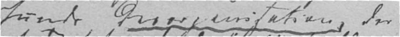funds Desorganisation, der
